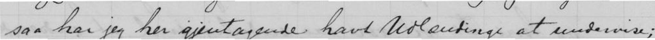saa har jeg her gjentagende havt Udlændinge at undervise;
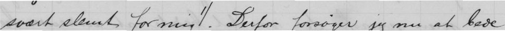svært slemt for mig1) Derfor forsøger jeg nu at bede
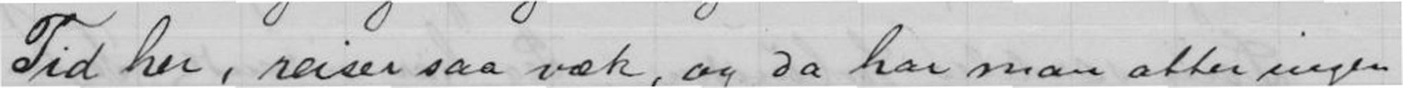Tid her, reiser saa væk, og da har man atter ingen
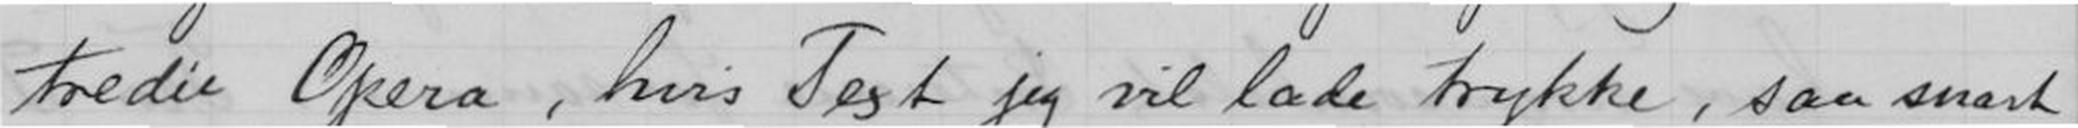tredie Opera, hvis Text jeg vil lade trykke, saa snart
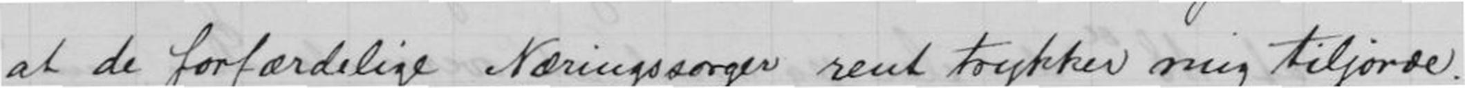at de forfærdelige Næringssorger rent trykker mig tiljorde.
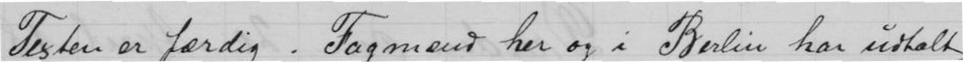Texten er færdig. Fagmænd her og i Berlin har udtalt
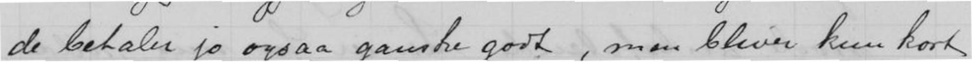de betaler jo ogsaa ganske godt, men bliver kun kort
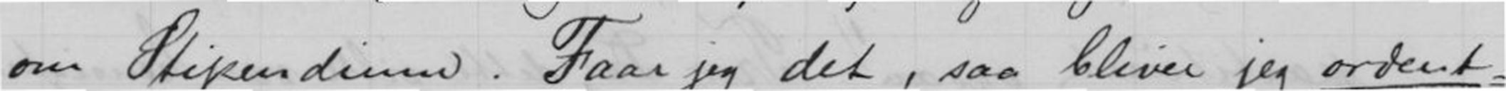om Stipendium. Faar jeg det, saa bliver jeg ordent-
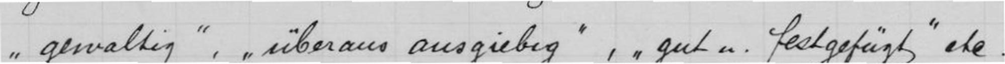gewaltig, überaus ausgiebig, gut u. festgefügt etc.
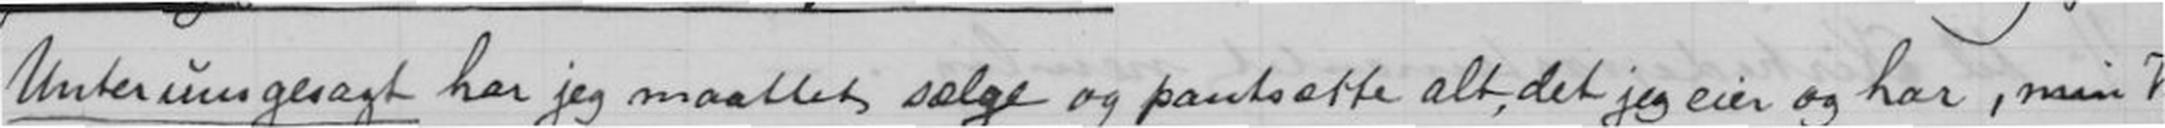1) Unterunsgesagt har jeg maatter selge og pansætte alt det jeg eier og har, min Violin ogsaa.
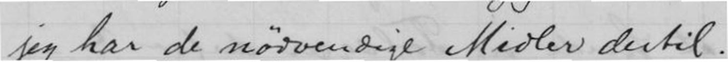jeg har de nødvendige Midler dertil. -
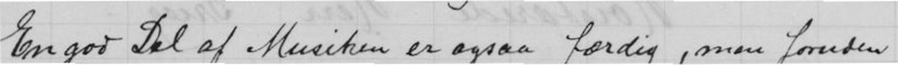En god del af Musiken er ogsaa færdig, men foruden
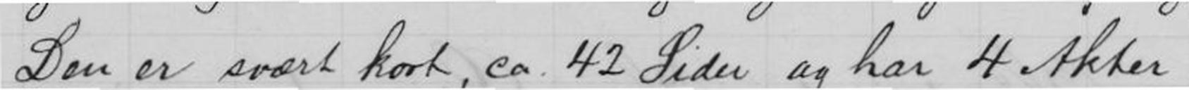Den er svært kort, ca 42 Sider og har 4 Akter. -
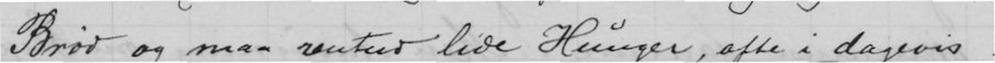Brød og maa rentud lide Hunger, ofte i dagevis. -
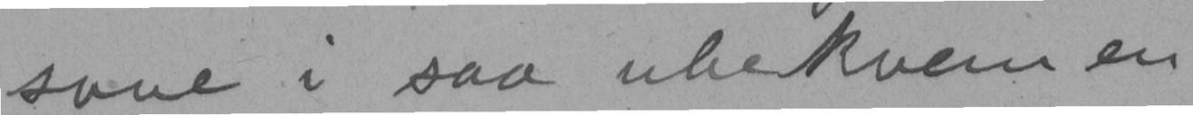sove i saa ubekvem en
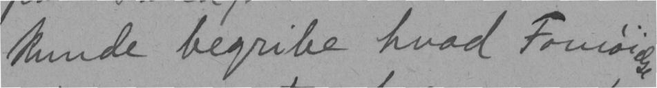kunde begribe hvad Fornøielse
